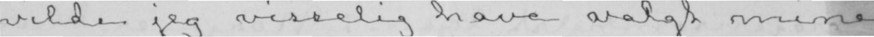vilde jeg visselig have valgt mine
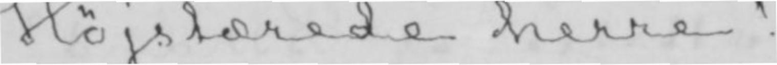Højstærede herre!
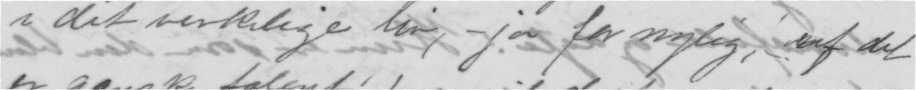i det virkelige liv, - ja for nylig, (uf det
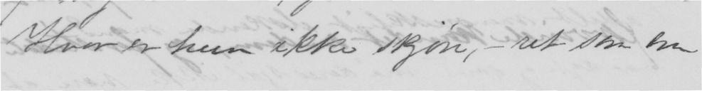Hvor er hun ikke skjøn, - ret som om
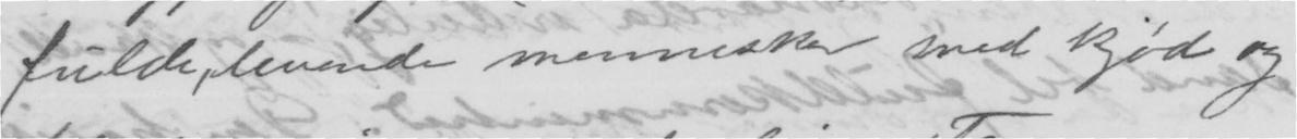fulde, levende mennesker med kjød og
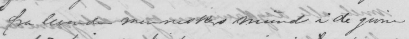fra levende menneskers mund i de givne
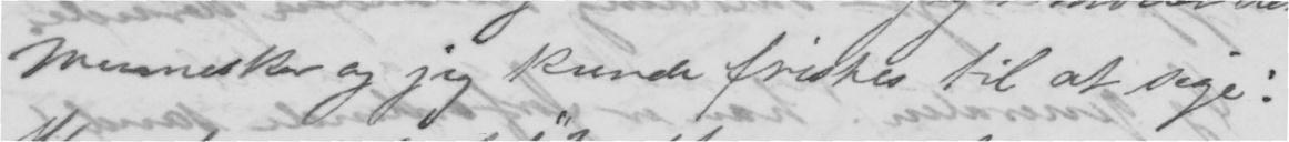mennesker og jeg kunde fristes til at sige:
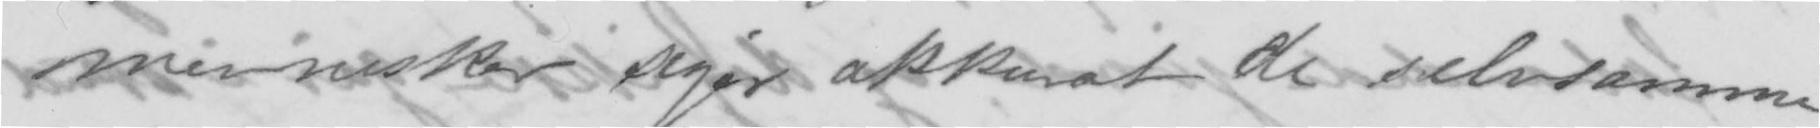mennesker siger akkurat de selvsamme
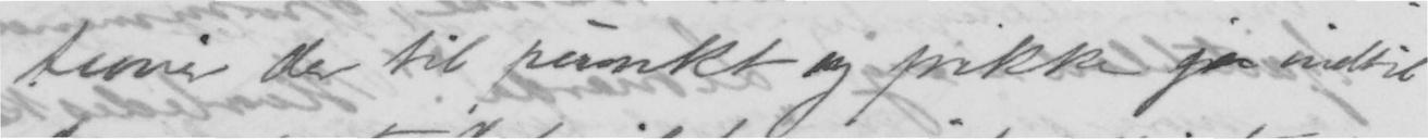tioner der til punkt og prikke ja indtil
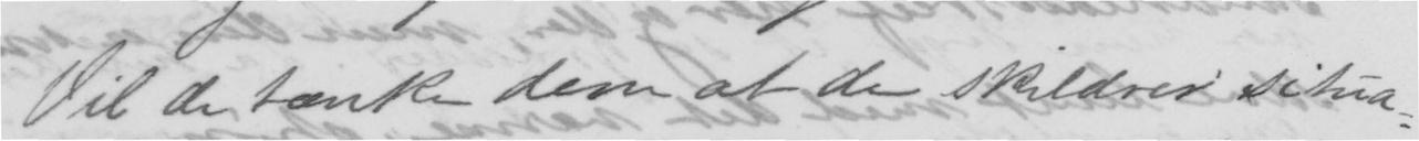Vil de tænke dem at de skildrer situa-
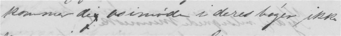kommer de os imøde i deres bøger, ikke
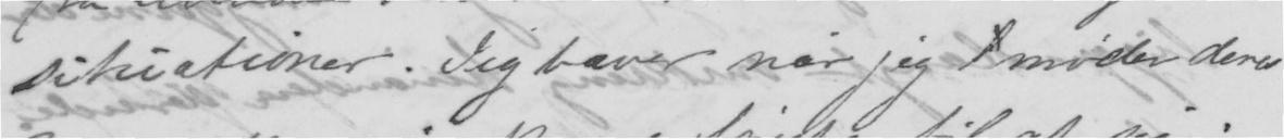situationer. Jeg bæver når jeg møder deres
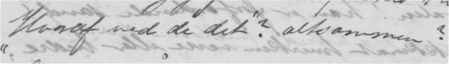"Hvoraf ved de det"? altsammen?
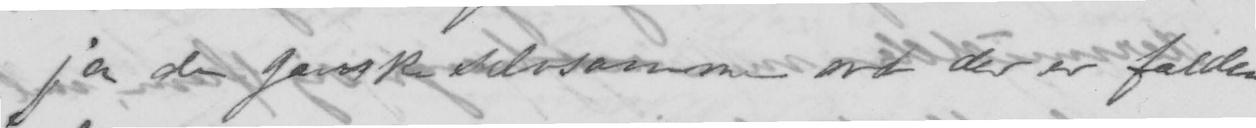ja de ganske selvsamme ord der er falden
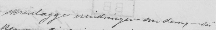skrinlægge erindringen om dem, - så
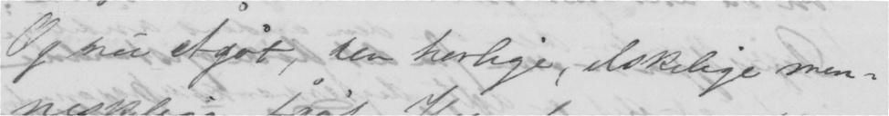Og nu Ågåt, den herlige, elskelige men-
